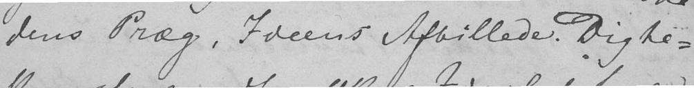dens Præg, Ideens Afbillede. Digte-
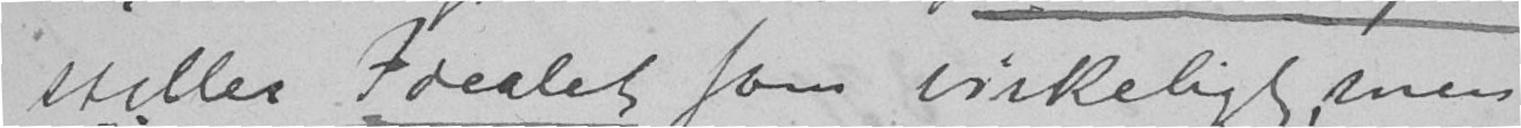stiller Idealet som virkeligt, men
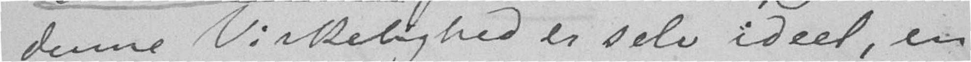denne Virkelighed er selv ideel, en
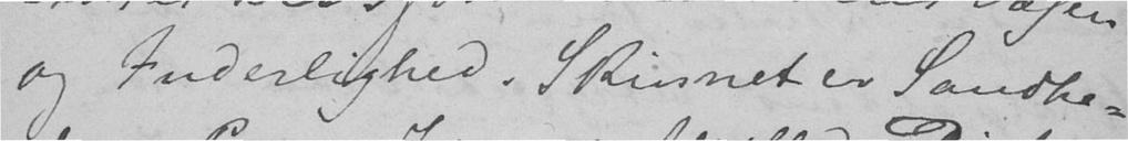og Inderlighed. Skinnet er Sandhe-
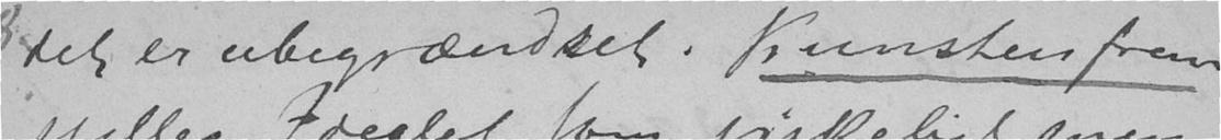det er ubegrændset. Kunsten frem-
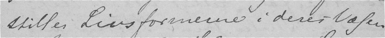stiller Livsformerne i deres Væsen
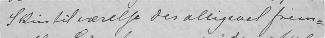Skintilværelse der alligevel frem-
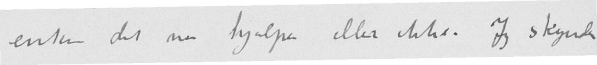enten det nu hjelper eller ikke. Jeg skynder
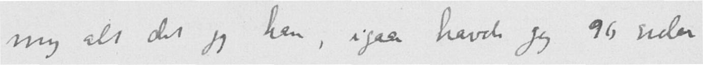mig alt det jeg kan, i gaar havde jeg 96 sider
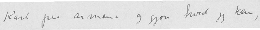Karl paa armen og gjøre hvad jeg kan,
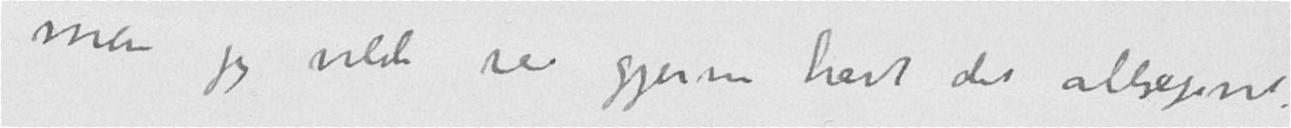men jeg vilde saa gjerne havt det alligevel.
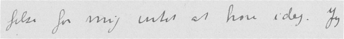felse for mig intet at høre i dag. Jeg
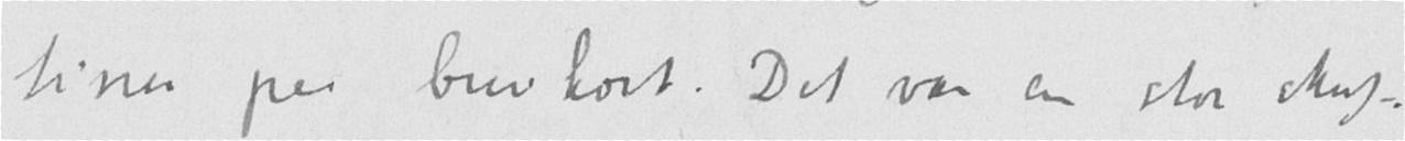tiner paa brevkort. Det var en stor skuf-
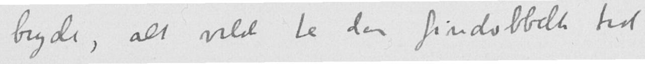bryde, alt vilde ta den firdobbelte tid
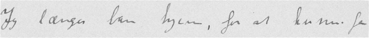Jeg længes bare hjem, for at kunne faa
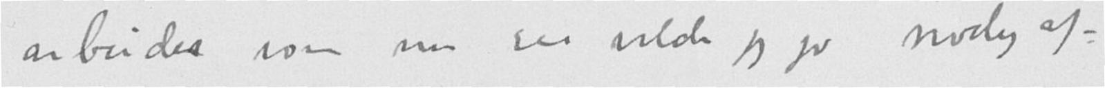arbeider som nu saa vilde jeg jo nødig af-
